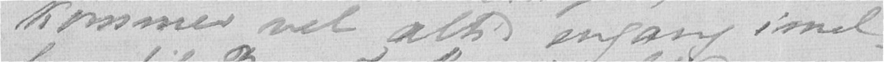kommer vel altid engang imel-
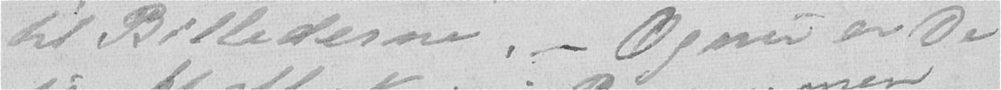til Billederne. - Og nu er De
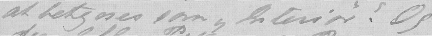at betegnes som "Interiør"? Og
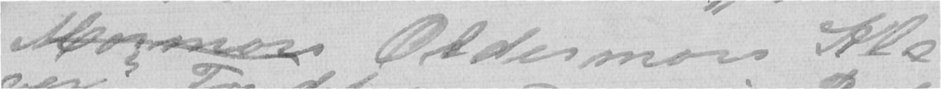Mormors Oldermors Kla-
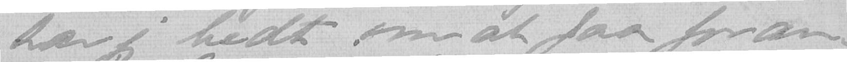har jeg bedt om at faa foran-
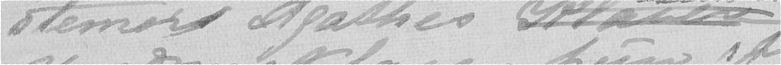stemors Agathes Klaver
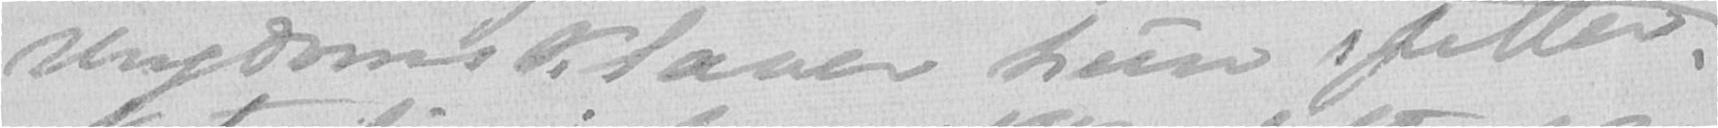Ungdomsklaver hun spiller.
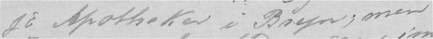jo Apotheker i Bryn; men
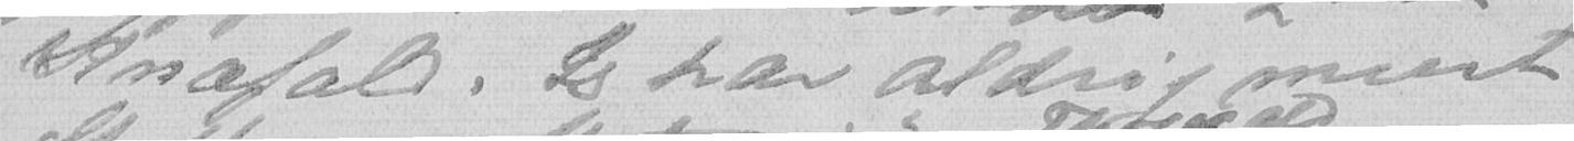Knæfald. Jeg har aldrig ment
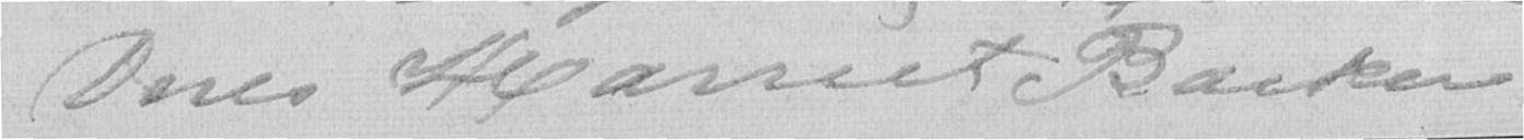Deres Harriet Backer
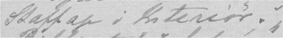Staffage i Interiør. "
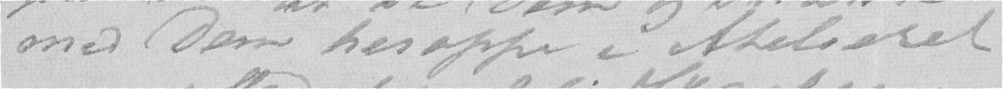med Dem heroppe i Atelieret
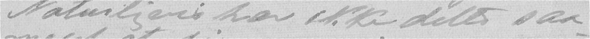Naturligvis har ikke dette saa
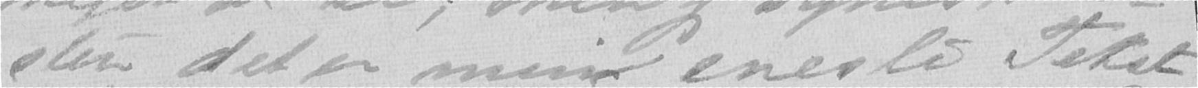sten det er min eneste Tekst
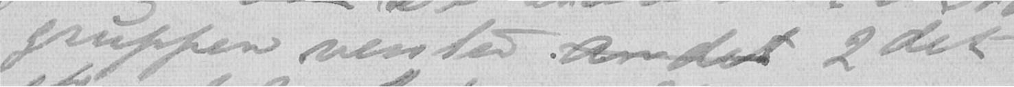gruppen venter andet 2det
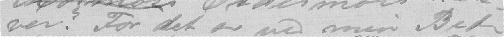ver". For det er ved min Bed-
